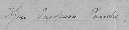Kjære Professor Paasche,
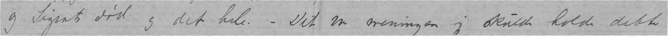og Sigvats død og det hele. – Det var meningen jeg skulde holde dette
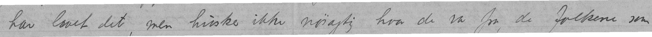har lavet det, men husker ikke nøiagtig hvor de var fra, de folkene som
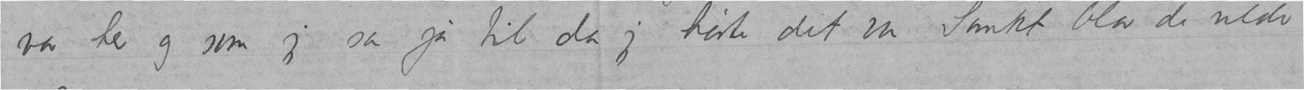var her og som jeg sa ja til da jeg hørte det var Sankt Olav de vilde
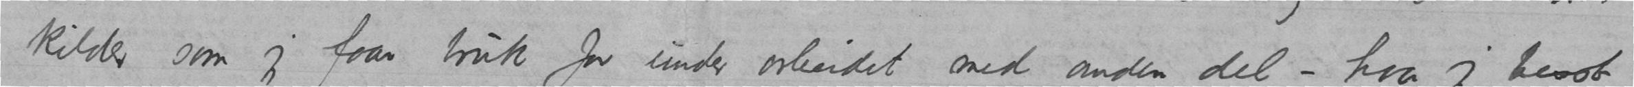kilder som jeg faar bruk for under arbeidet med anden del – hvor jeg besst
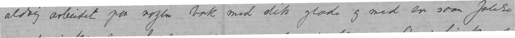aldrig arbeidet paa nogen bok med slik glæde og med en saan følelse
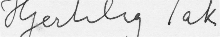Hjertelig Tak
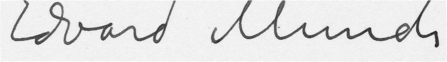Edvard Munch
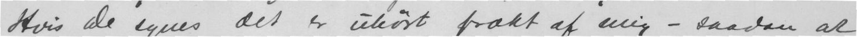Hvis De synes det er uhørt frækt af mig - saadan at
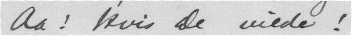Aa ! hvis De vilde !
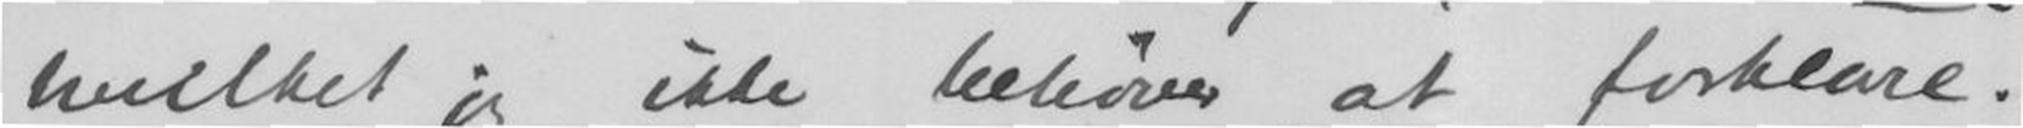hvilket jeg ikke behøver at forklare.
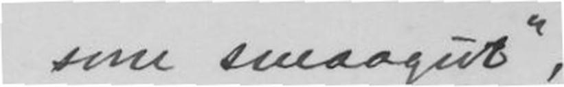som smaagut",
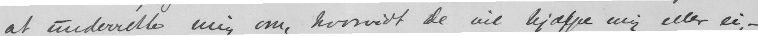at underrette mig om, hvorvidt De vil hjælpe mig eller ei, -
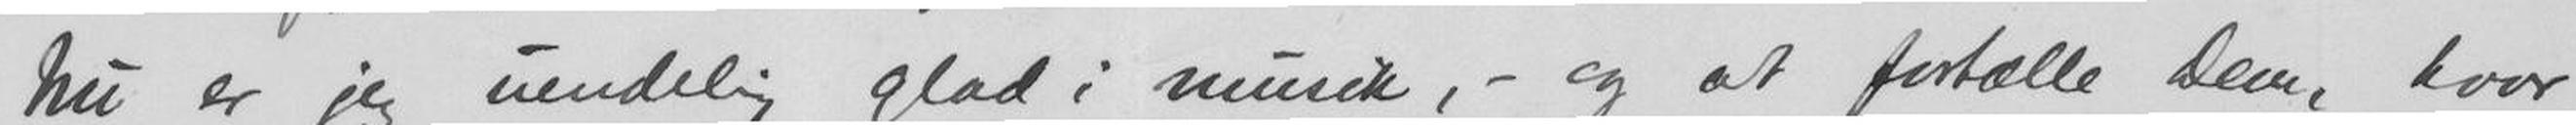Nu er jeg uendelig glad i musik, - og at fortælle Dem, hvor
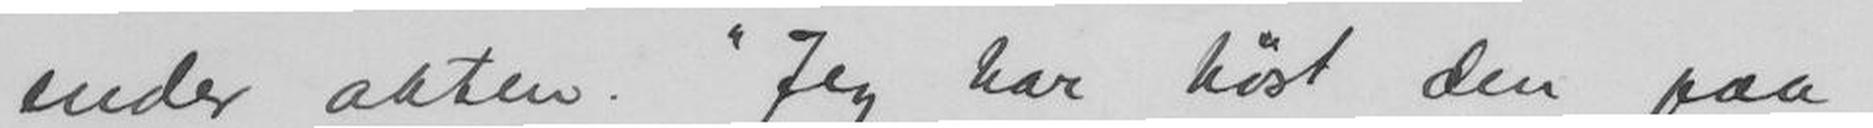andre akten. "Jeg har hørt den paa
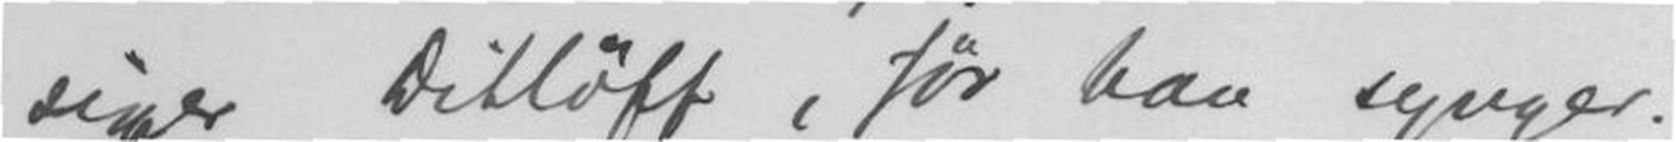siger Ditløff, før han synger
